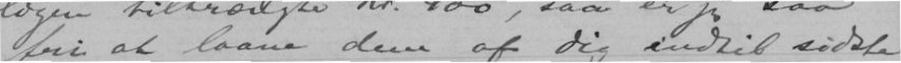fri at laane dem af Dig indtil sidste
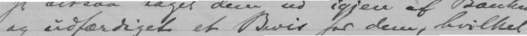og udfærdiget et Bevis for dem, hvilket
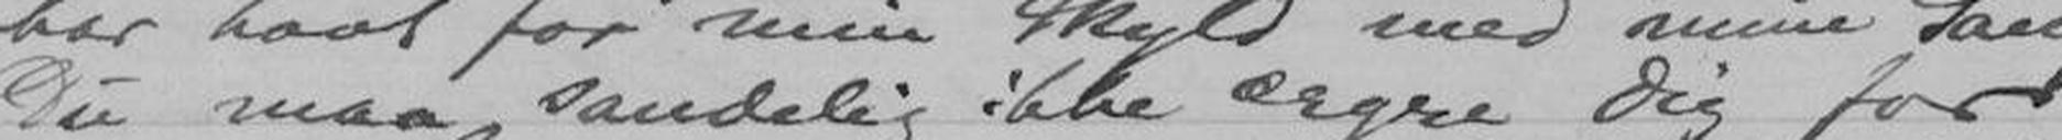Du maa sandelig ikke ærgre Dig for
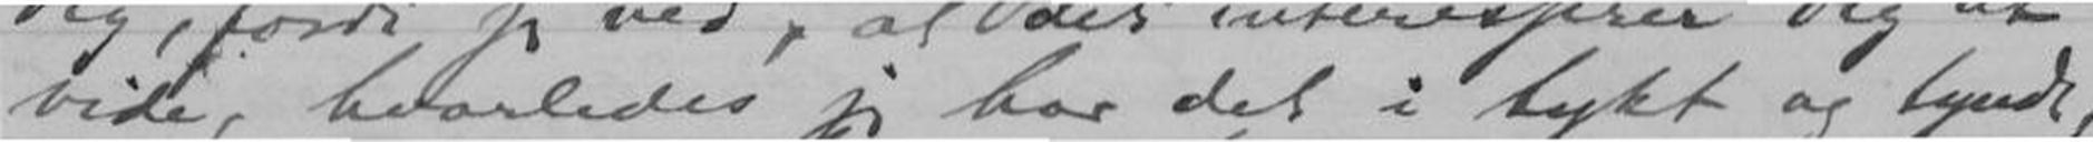vide, hvorledes jeg har det i tykt og tyndt,
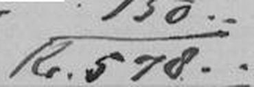Kr. 578.-
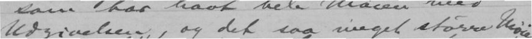Udgivelsen, og det saa meget større Møie
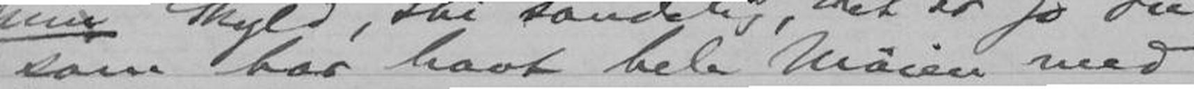som har havt hele Møien med
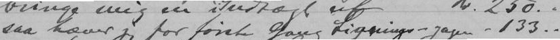saa hæver jeg for første Gang Lignings-gagen " 133.-
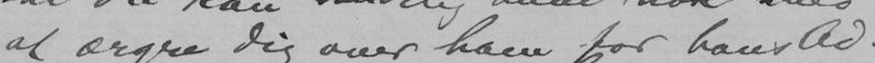at ærgre Dig over ham for hans Ad-
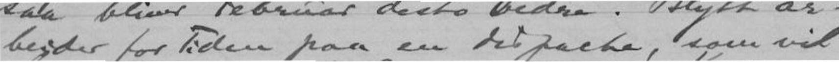beider for Tiden paa en Dispache, som vil
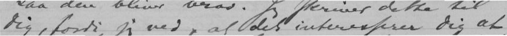Dig, fordi jeg ved, at det interesserer dig at
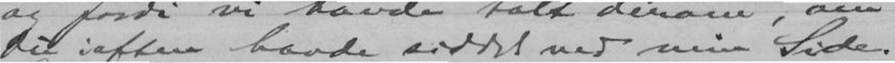Du iaften havde siddet ved min Side.
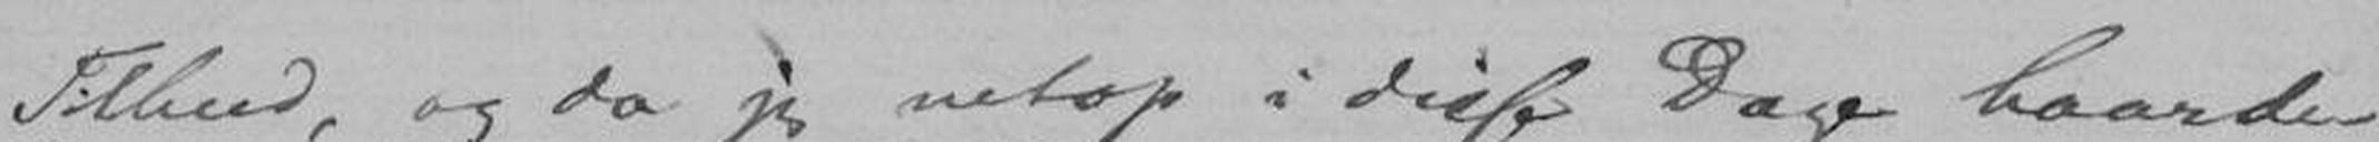Tilbud, og da jeg netop i disse Dage haarde-
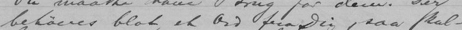behøves blot et Ord fra Dig, saa skul-
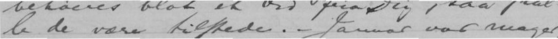le de være tilstede. - Januar var mager,
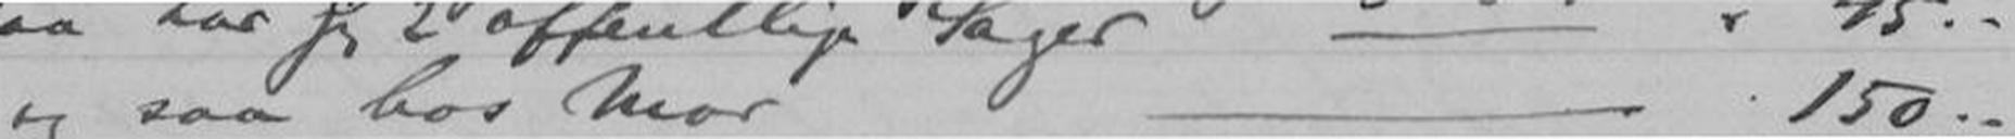og saa hos Mor -------------------------------- " 150.-
